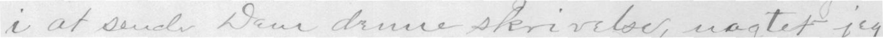i at sende Dem denne skrivelse, uagter jeg
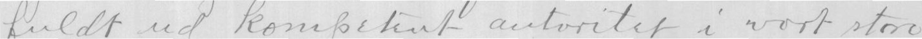fuldt ud, kompetent autoritet i vort store
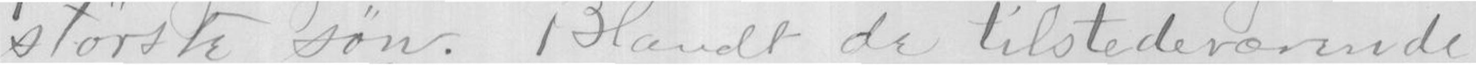største søn. Blandt de tilstedeværende
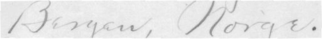Bergen, Norge
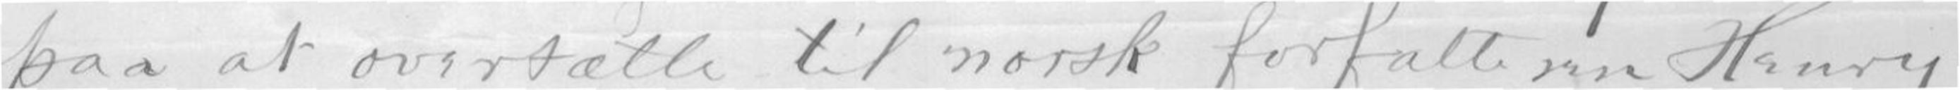paa at oversætte til norsk forfatter Henry
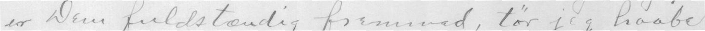er Dem fuldstændig fremmad, tør jeg haabe
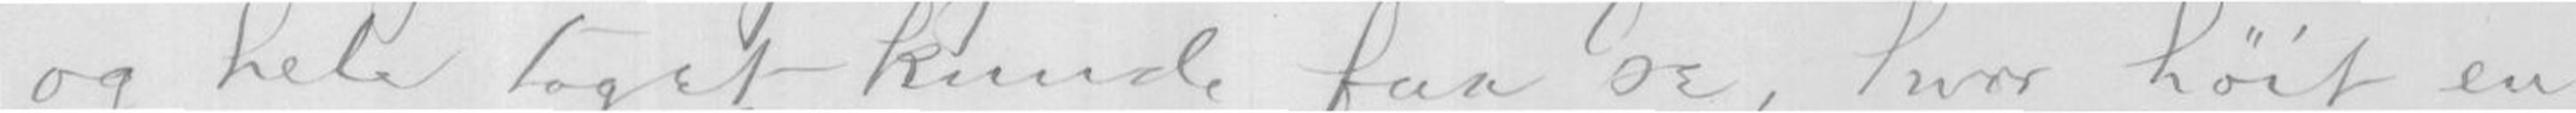og hele taget kunde faa os, hvor høit en
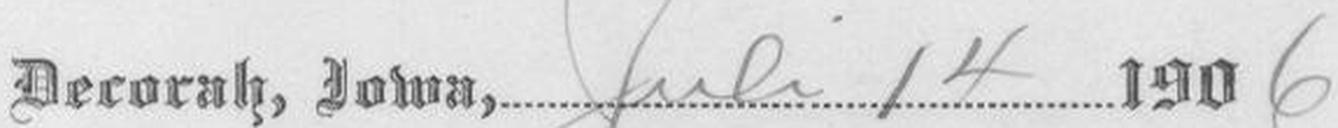Decorah, Iowa, Juli 14 1906
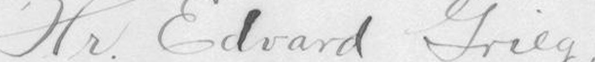Hr. Edvard Grieg,
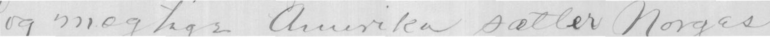og megtige Amerika sætter Norges
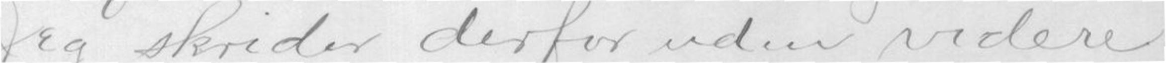Jeg skrider derfor uden videre
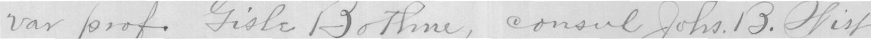var prof. Gisle Bothne, consul Johs. B. Hist
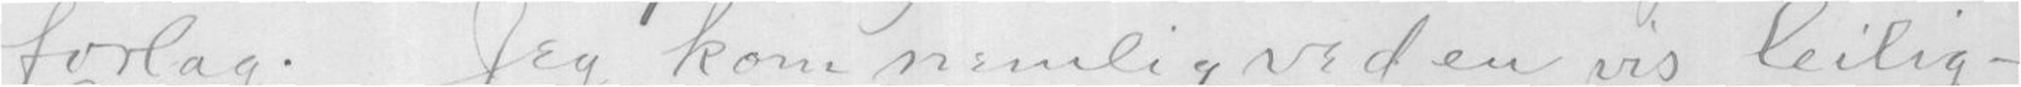forlag. Jeg kom nemlig ved en vis leilig-
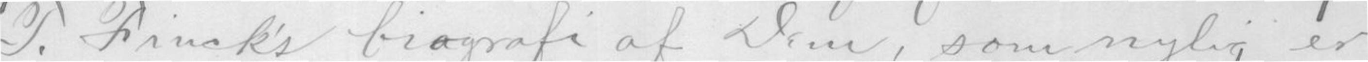T.Finck's biografi af Dem, som nylig er
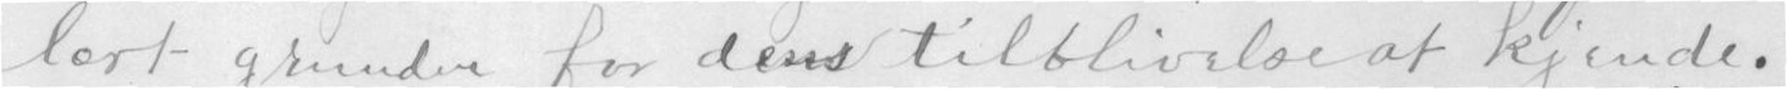lært grunden for dens tilblivelse at kjende.
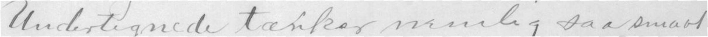Undertegnede tænker nemlig saa snart
